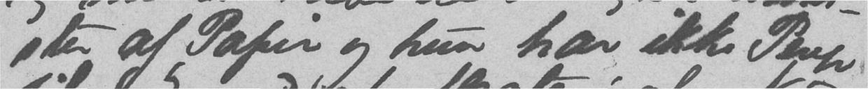ster af Papir og hun har ikke Penge
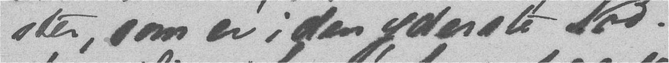ster som er i den yderste Nød.
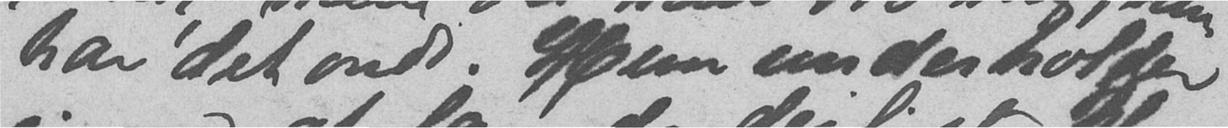har det ondt. Hun underholder,
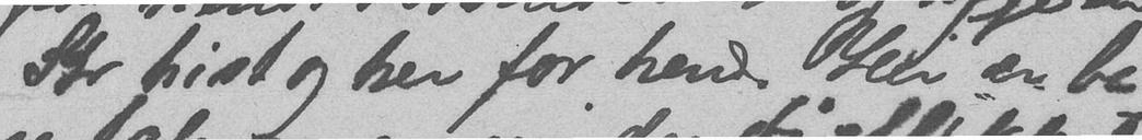Kr hist og her for hende. Her er ba-
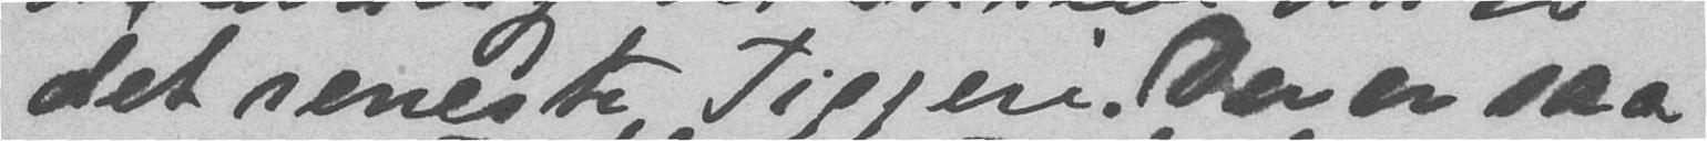det reneste Tiggeri. Der er saa
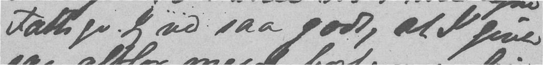Fattige. Jeg ved saa godt, at I give
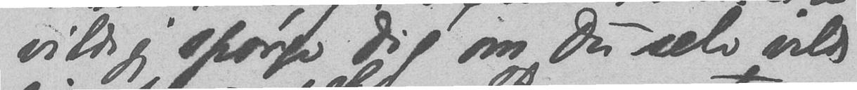vilde jeg spørge dig om Du selv vilde
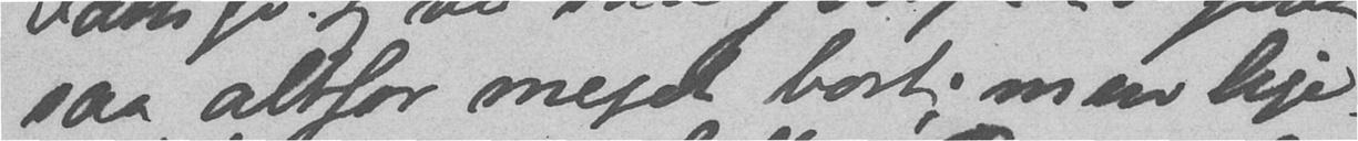saa altfor meget bort, men lige-
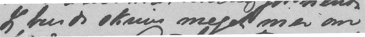Jeg burde skrive meget mer om
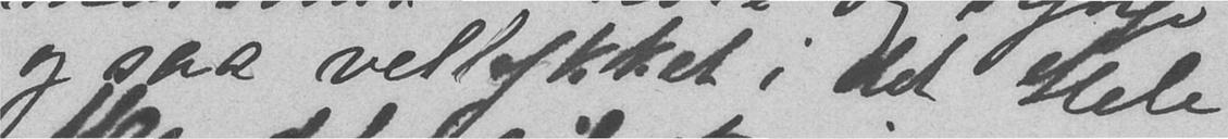og saa vellykket i det Hele
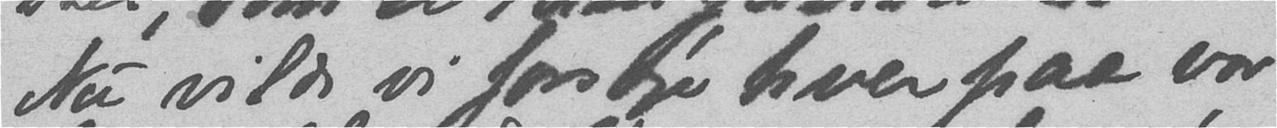Nu vilde vi  hver paa vor-
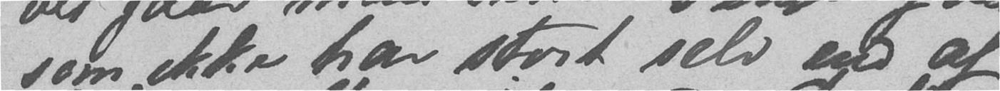som ikke har stort selv end af
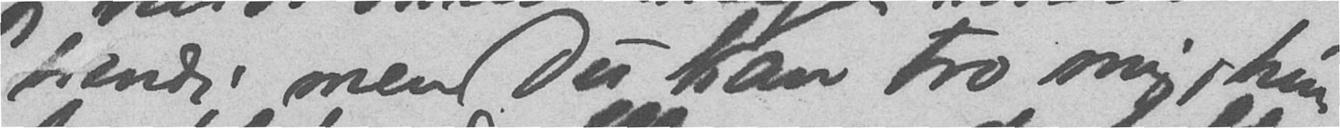hende; men Du kan tro mig, hun
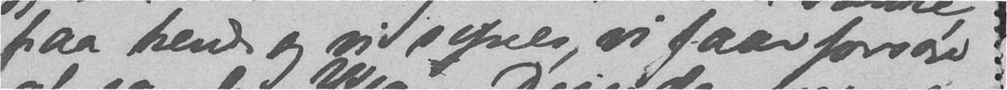paa hende og vi synes vi faar forsøge
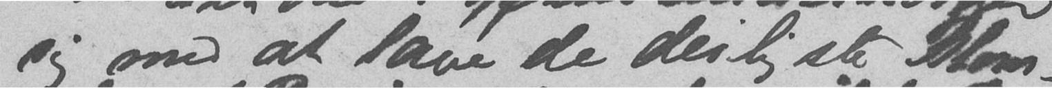sig med at lave de deiligste Blom-
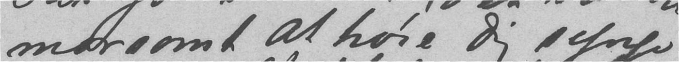morsomt at høre deg synge
